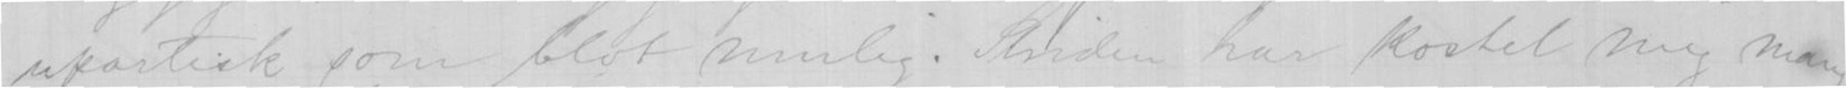upartisk som blot mulig. Striden har kostet mig mangen
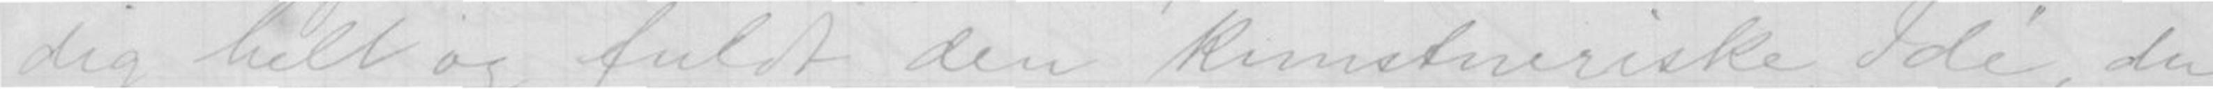dig helt og fuldt den kunstneriske Idé, du
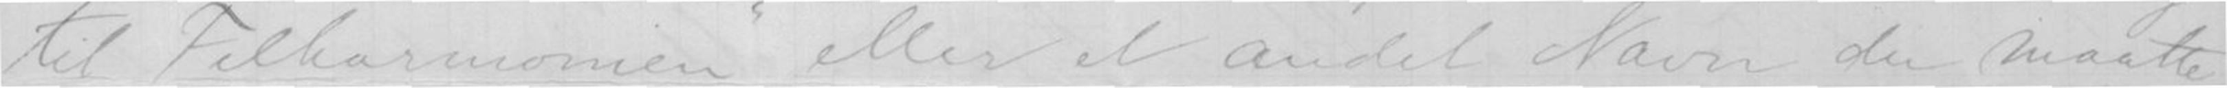til Filharmonien eller et andet Navn du maatte
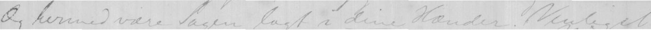Og hermed være Sagen lagt i dine Hænder. Venligst
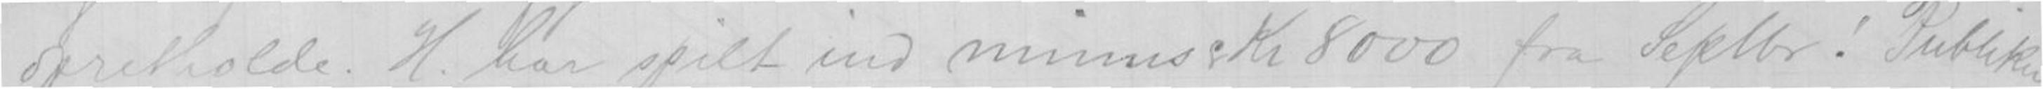opretholde. H. har spilt ind minus Kr 8000 fra Septbr! Publikum
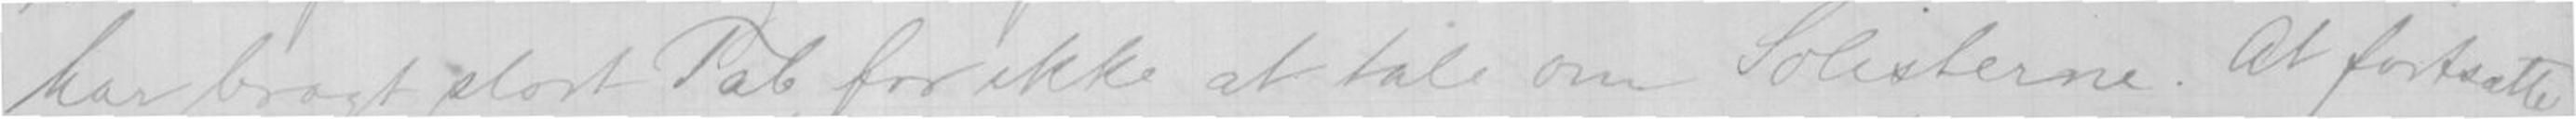har bragt stort Tab, for ikke at tale om Solisterne. At fortsætte
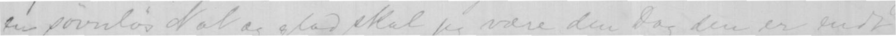en søvnløs Nat og glad skal jeg være den Dag den er endt
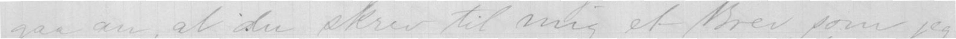gaa an, at du skrev til mig et Brev, som jeg
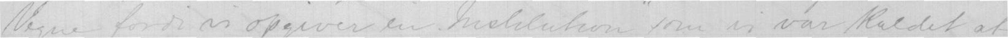Vegne fordi vi opgiver en Institution som vi var kaldet at
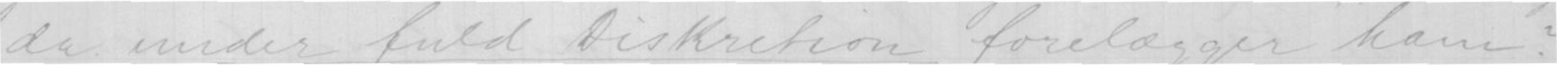da under fuld Diskretion forelægger ham?
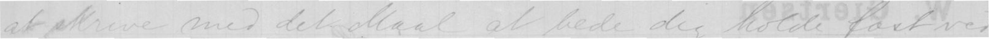at skrive med det Maal at bede dig holde fast ved
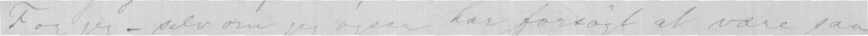F og jeg - selv om jeg ogsaa har forsøgt at være saa
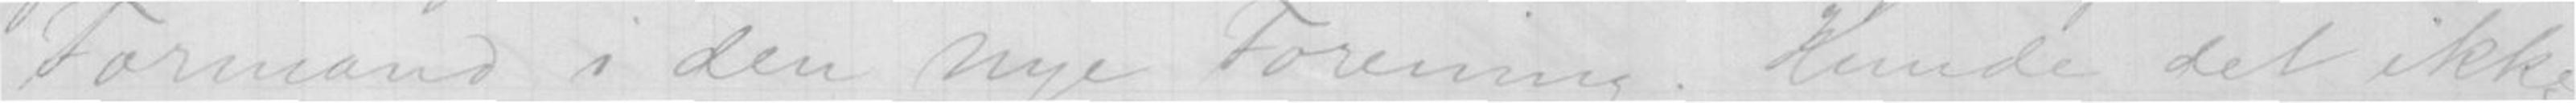Formand i den nye Forening. Kunde det ikke
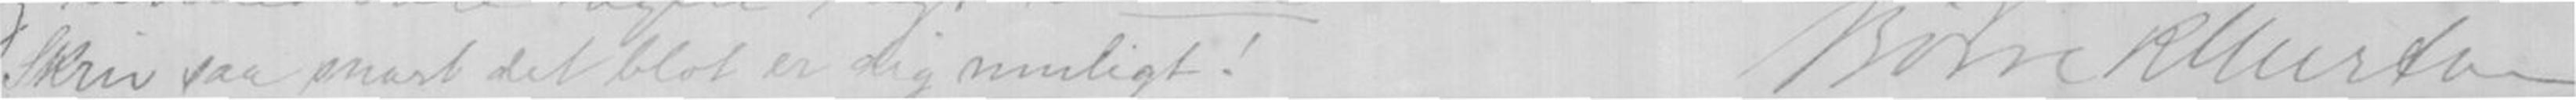Skriv saa snart det blot er dig muligt! Børre R Giertsen
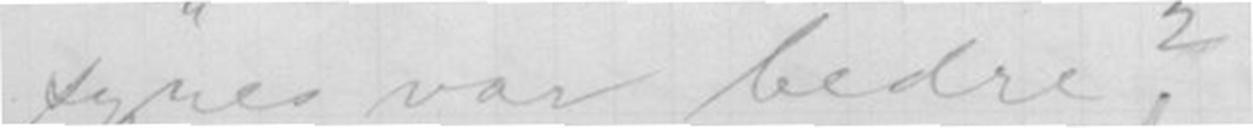synes var bedre?
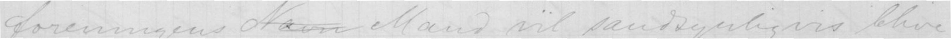foreningens Navn Mand vil sandsynligvis blive
